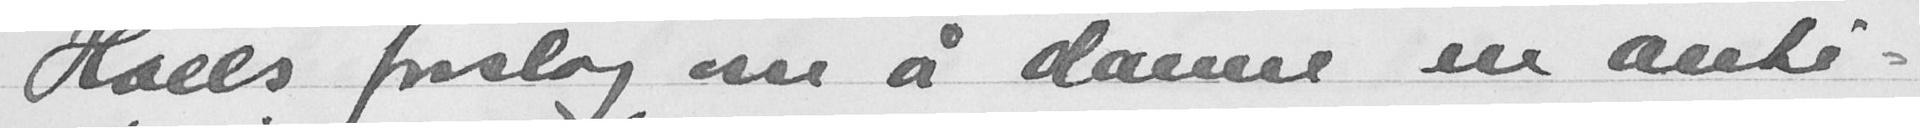Hoels forslag om å danne en anti-
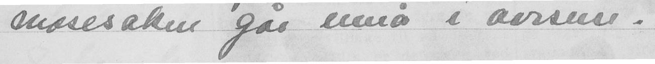mosesaken går ennå i avisene.
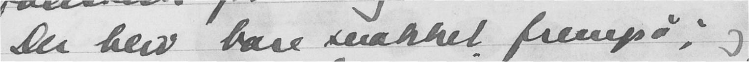Der blev bare snakket frempå; og
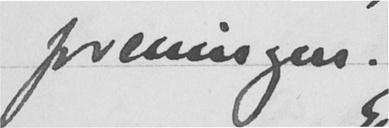foreningen.
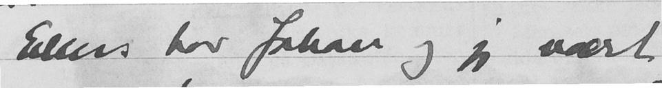Ellers har Johan og jeg vært
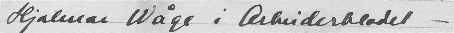Hjalmar Wåge i Arbeiderbladet -
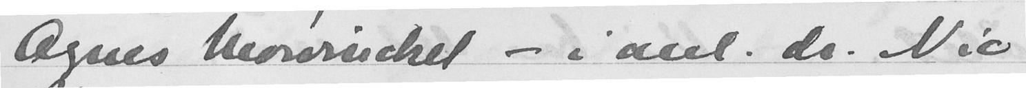Agnes Mowinckel - i anl. dr. Nic
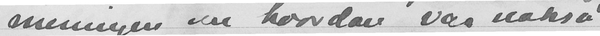meningen om hvordan var nokså
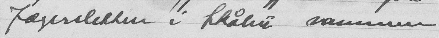Jægersletten i Skåbu sammen
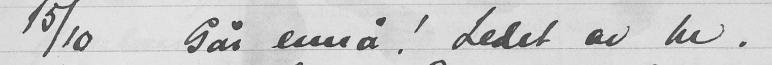15/10 Går ennå! Ledet av hr.
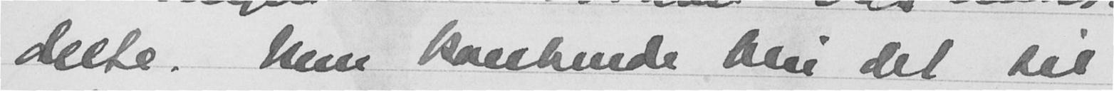delte. Men kanhende blir det til
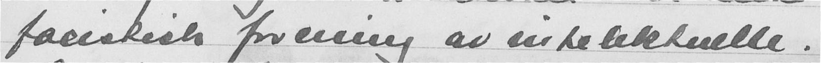facistisk forening av intelektuelle.
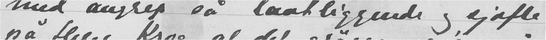med angrep så lavtliggende og sjofle
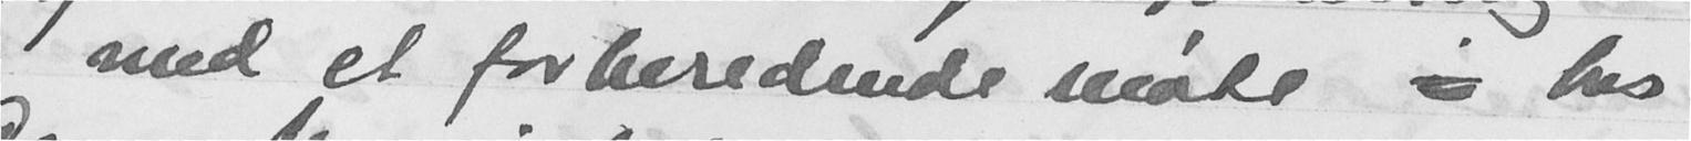med et forberedende møte  hos
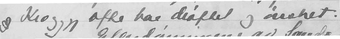og Krog jo ofte har drøftet og ønsket.
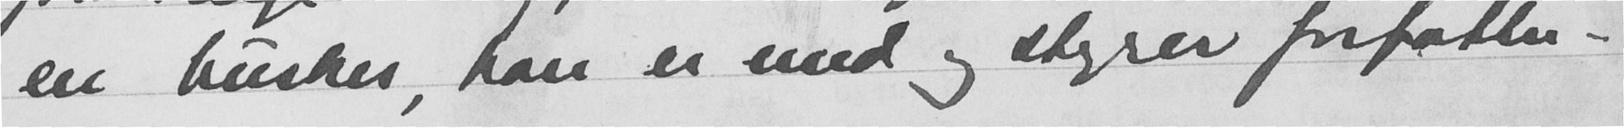en husker, han er med og styrer forfatter-
